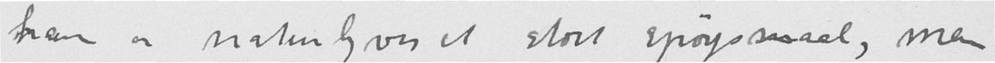ham er naturligvis et stort spørgsmaal, men
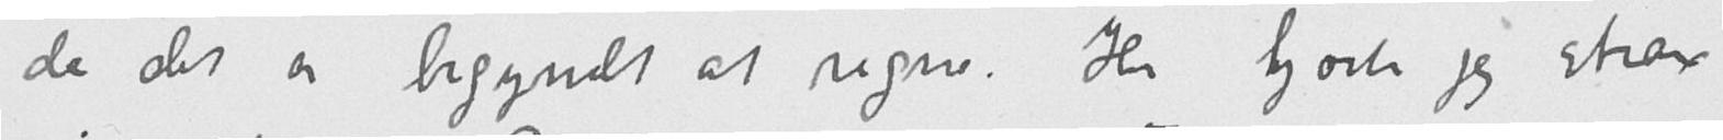da det er begyndt at regne. Her kjørte jeg strax
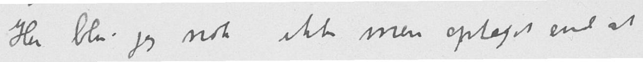Her blir jeg nok ikke mere optaget end at
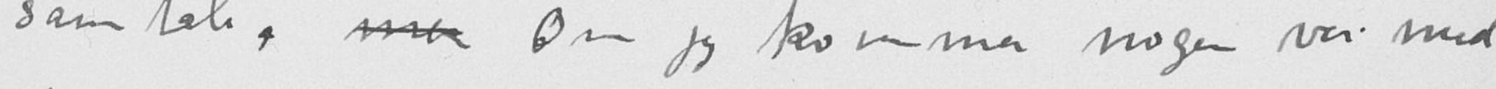samtale, men Om jeg kommer nogen vei med
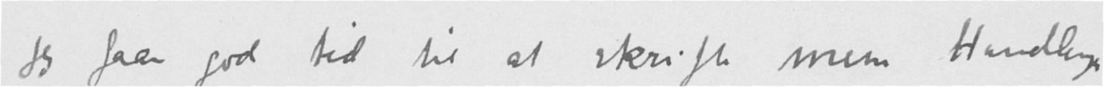jeg faar god tid til at skrifte mine Handlinger
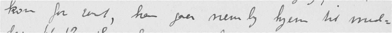kom for sent, han gaar nemlig hjem til mid-
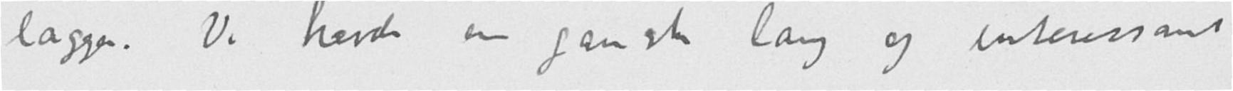lægger. Vi havde en ganske lang og interessant
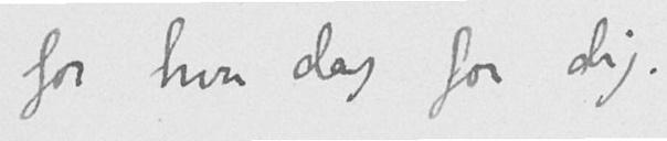for hver dag for dig.
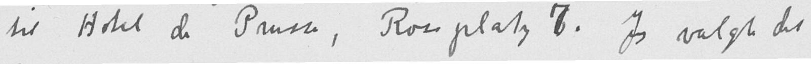til Hotel de Prusse, Rossplatz 7. Jeg valgte det
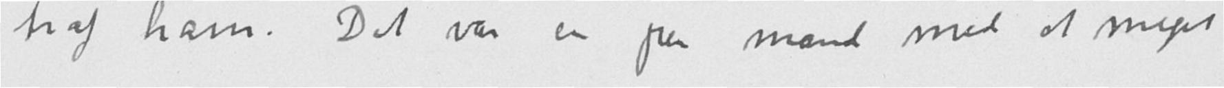traf ham. Det var en pen mand med et meget
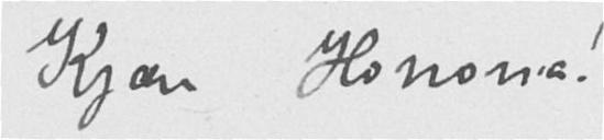Kjære Honoria!
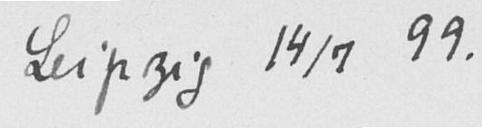Leipzig 14/7 99.
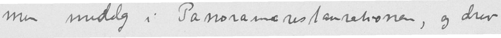min middag i Panoramarestaurationen, og drev
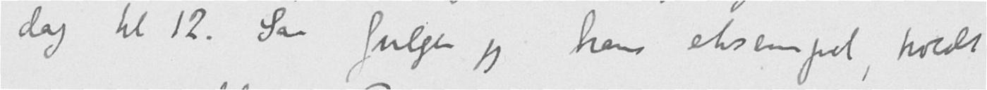dag kl 12. Saa fulgte jeg hans eksempel, holdt
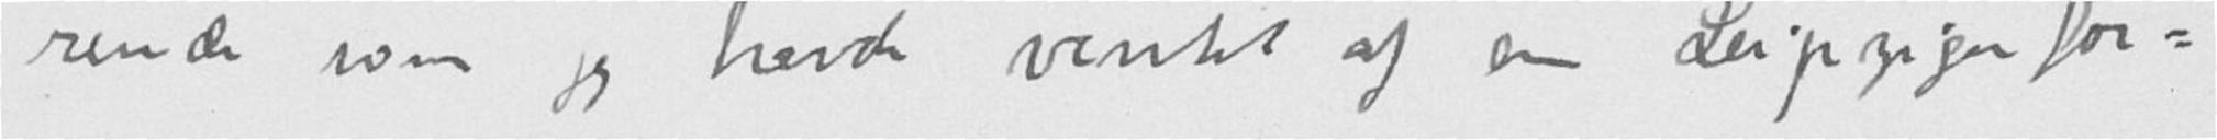rende som jeg havde ventet af en Leipzigerfor-
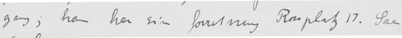gang; han har sin forretning Rossplatz 17. Saa
